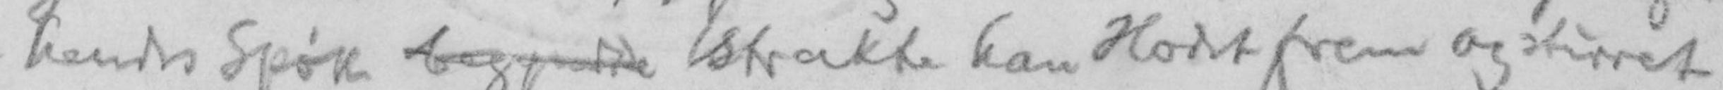hendes Spøk begyndte strakte han Hodet frem og stirret
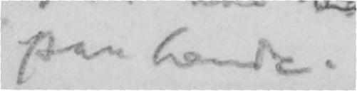paa hende.
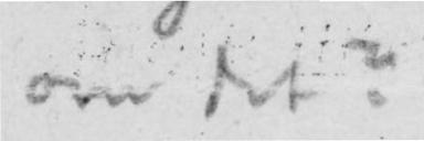om det?
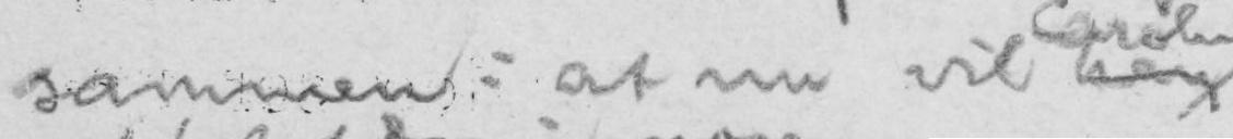sammen: at nu vil han
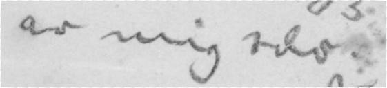av mig selv.
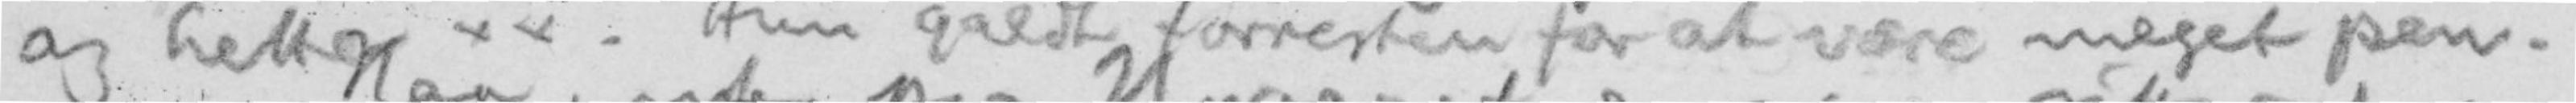og hette x x. Hun galdt forresten for at være meget pen.
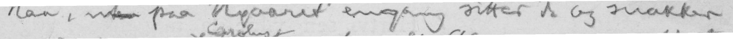Naa, uten paa Nyaaret engang sitter de og snakker
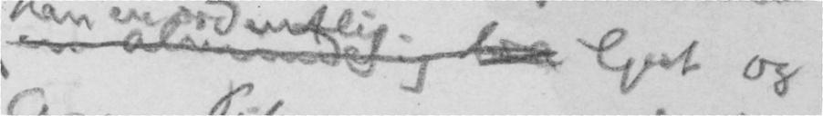en almindelig og bra Gut og
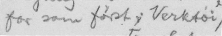for som først; Verktøi
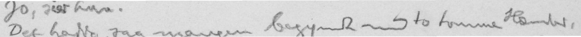Det hadde saa mangen begyndt med to tomme Hænder,
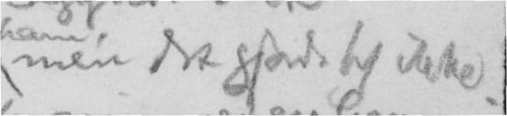men det gjorde h. ikke.
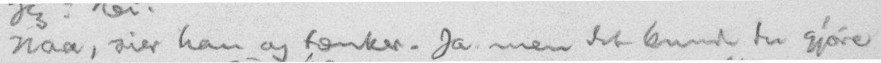Naa, sier han og tænker. Ja men det kunde du gjøre
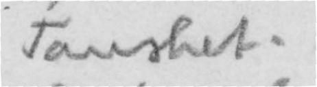Taushet.
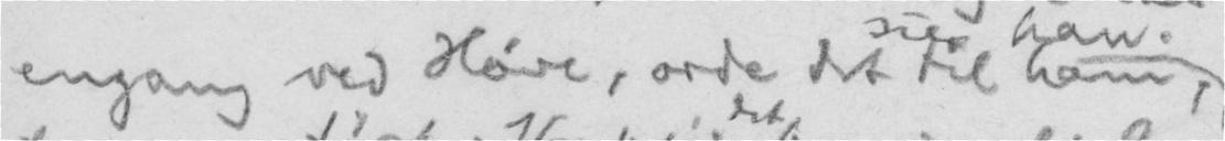engang ved Høve, orde det til ham,
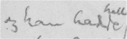og han hadde
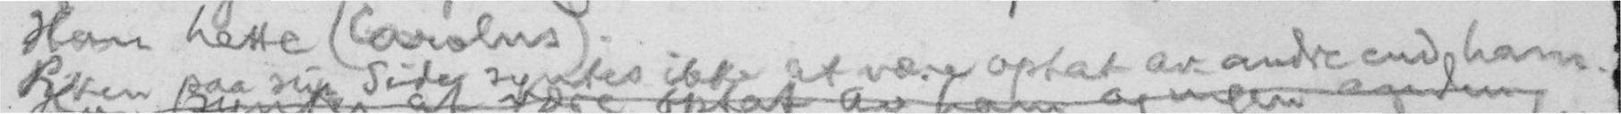Piken paa sin Side syntes ikke at være optat av andre end ham.
